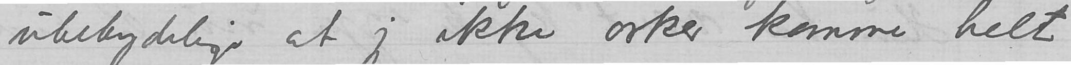ubetydelige at jeg ikke orker komme helt
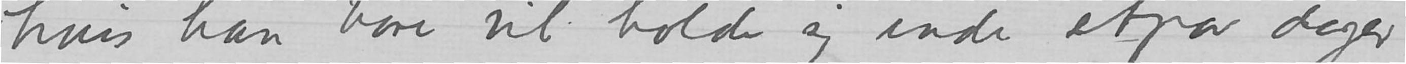hvis han bare vil holde sig inde etpar dager
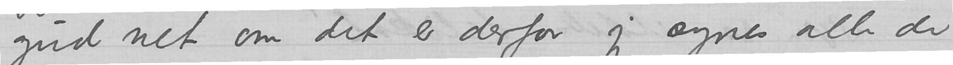– gud vet om det er derfor jeg synes alle de
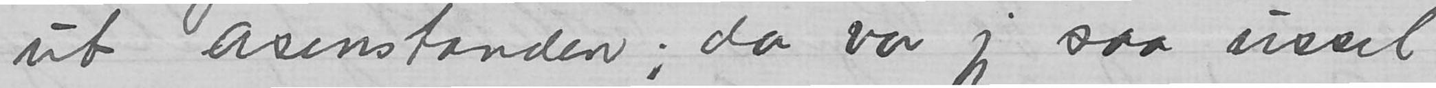ut <asenstanden>; da var jeg saa ussel
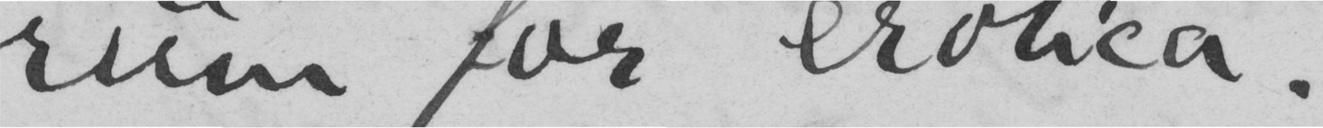rum for erotica.
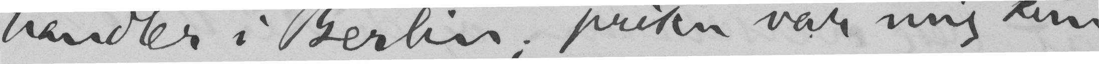handler i Berlin; prisen var mig kun
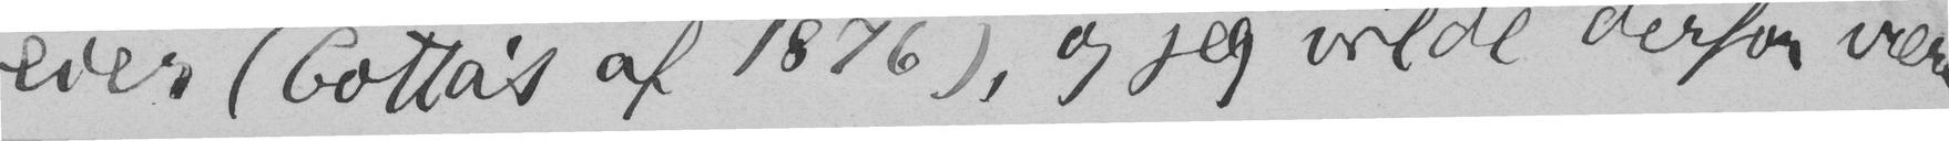eier (Cotta’s af 1876), og jeg vilde derfor være
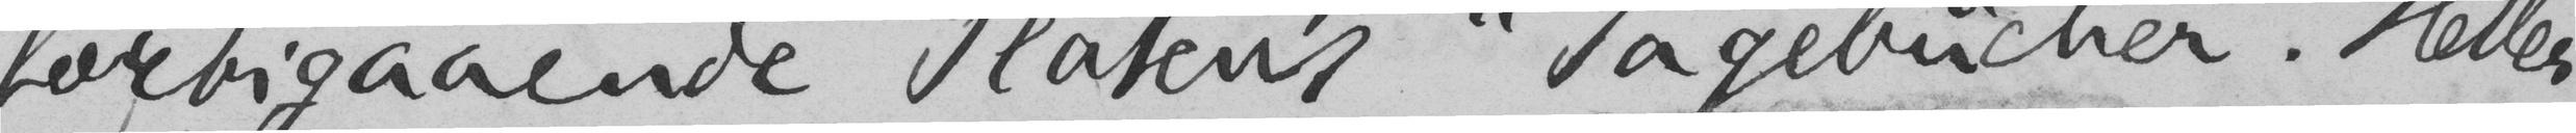forbigaaende Platens «Tagebücher». Heller
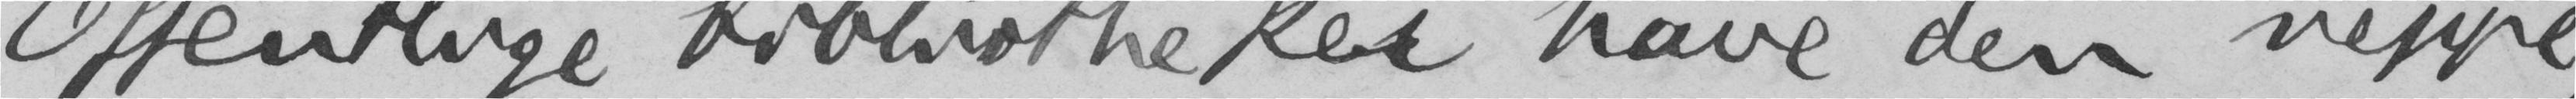Offentlige bibliotheker have den neppe,
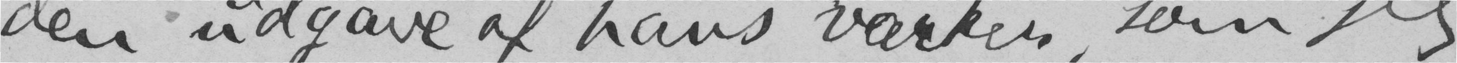den udgave af hans værker, som jeg
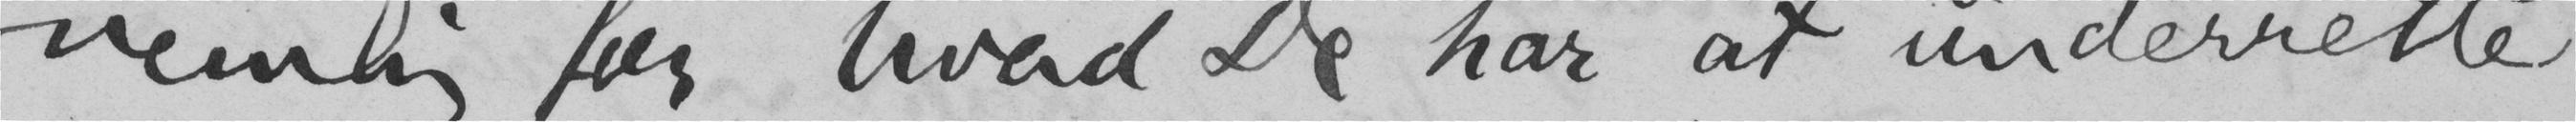nemlig for, hvad De har at underrette
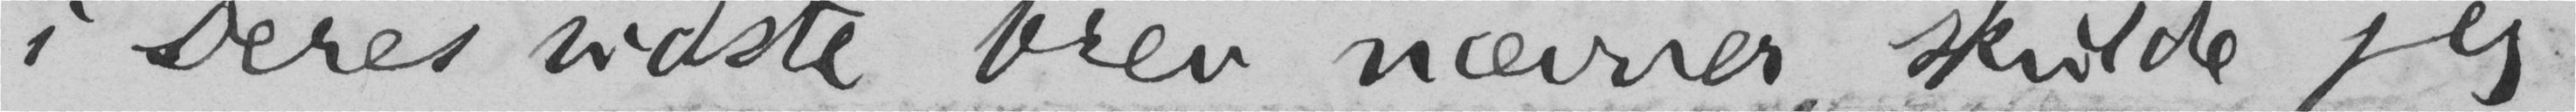i Deres sidste brev nævner, skulde jeg
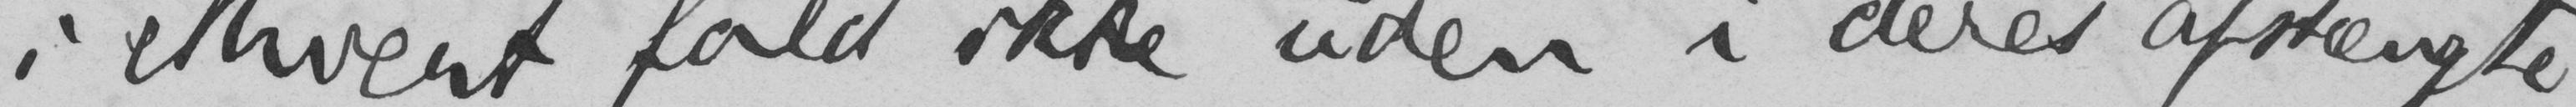i ethvert fald ikke uden i deres afstængte
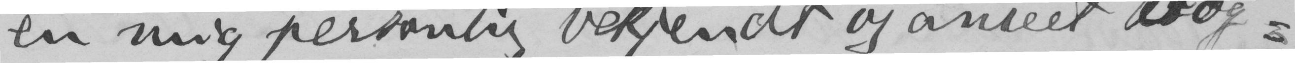en mig personlig bekjendt og anseet Bog-
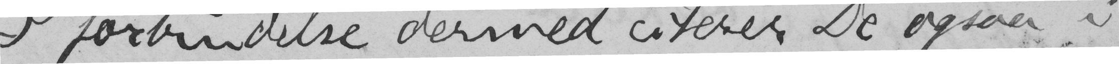I forbindelse dermed citerer De ogsaa i
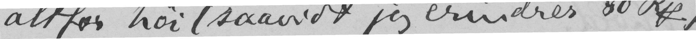altfor høi (saavidt jeg erindrer 80 R).
